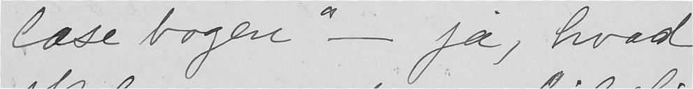læse bogen" - ja, hvad
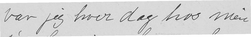var jeg hver dag hos min
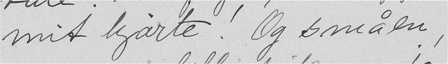mit hjærte! Og småen,
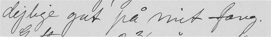dejlige gut på mit fang.
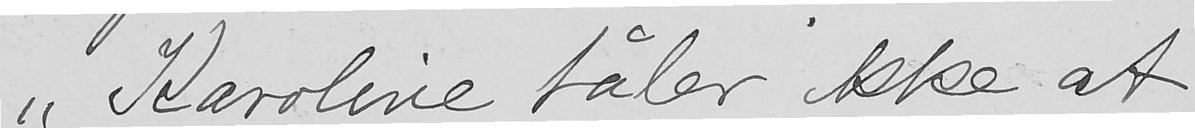"Karoline tåler ikke at
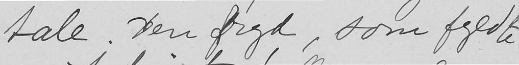tale. Den fryd, som fyldte
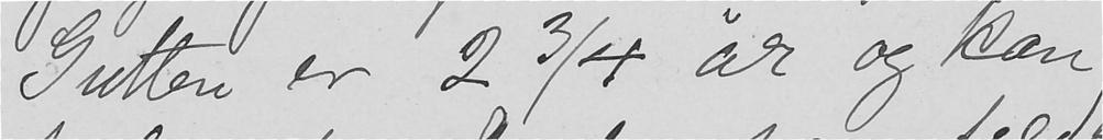Gutten er 2 3/4 år og kan
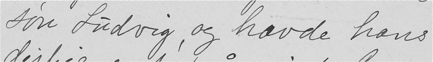søn Ludvig og havde hans
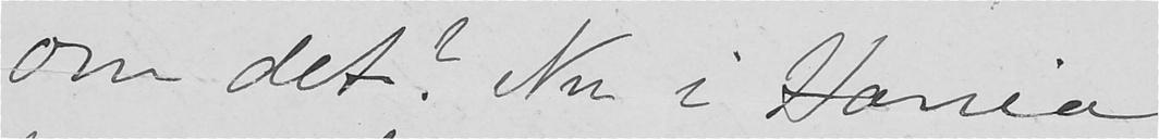om det? Nu i Xania
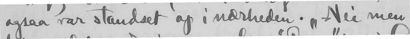ogsaa var standset op i nærheden. "Nei men
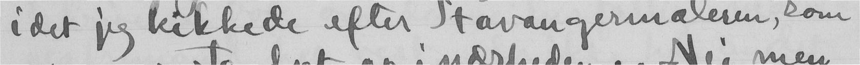i det jeg kikkede efter Stavangermaleren, som
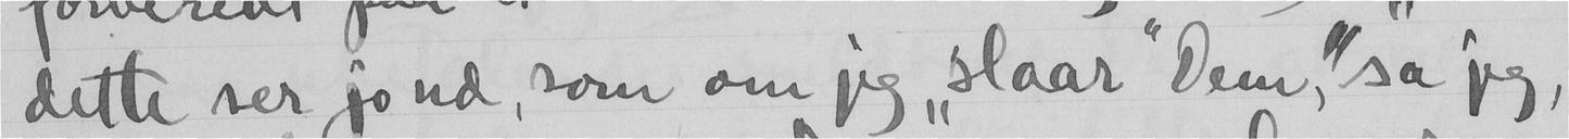dette ser jo ud, som om jeg "slaar" Dem", sa jeg,
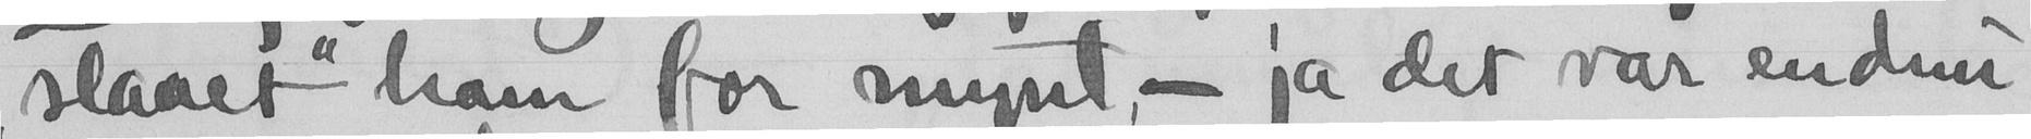"slaaet" ham for mynt, - ja det var endnu
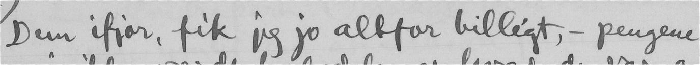Dem ifjor, fik jeg jo altfor billigt, - pengene
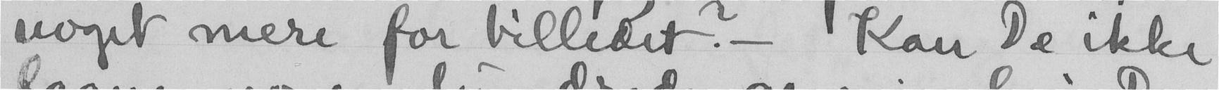noget mere for billedet? - Kan De ikke
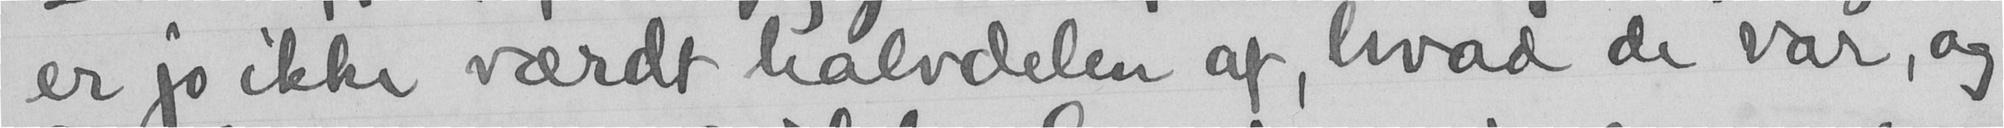er jo ikke værdt halvdelen af, hvad de var, og
